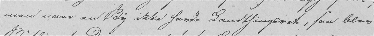men naar en By ikke havde Landthingsret, saa blev
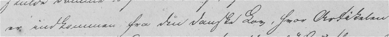er indkommen fra den danske Lov, hvor Artikelen
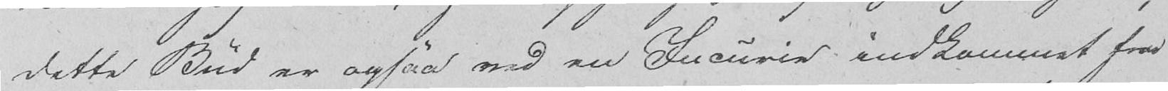dette Bud er ogsaa ved en Incurie indkommet fra
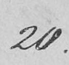20.
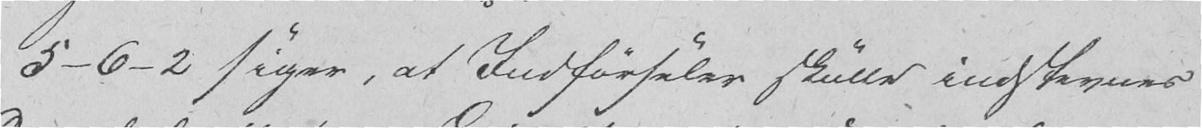5-6-2 siger, at Indførseler skulle indstevnes
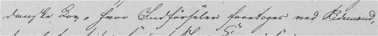danske Lov, hvor Indførseler foretoges ved Ridemænd,
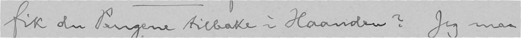fik du Pengene tilbake i Haanden? Jeg maa
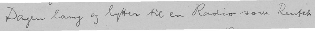Dagen lang og lytter til en Radio som Rentch
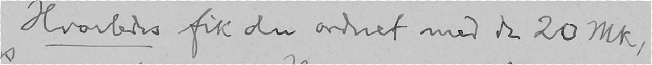Hvorledes fik du ordnet med de 20 Mk,
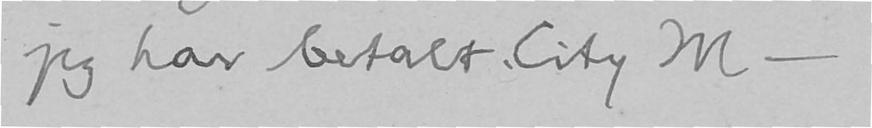jeg har betalt City M -
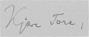Kjære Tore,
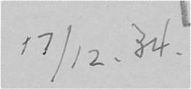17/12. 34.
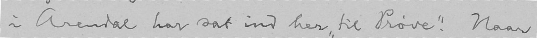i Arendal har sat ind her "til Prøve". Naar
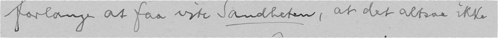forlange at faa vite Sandheten, at det altsaa ikke
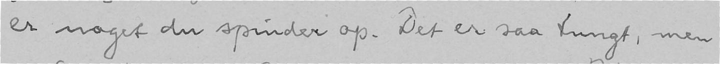er noget du spinder op. Det er saa tungt, men
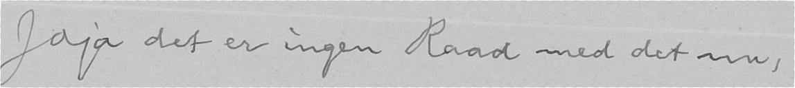Jaja det er ingen Raad med det nu,
